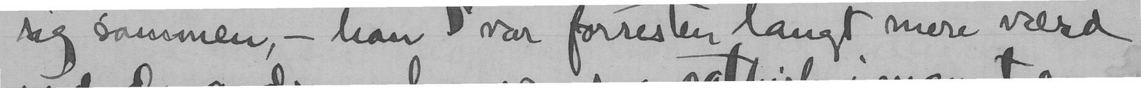sig sammen, - han var forresten langt mere værd
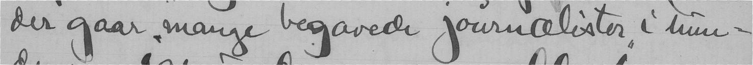der gaar mange begavede journalister i hun-
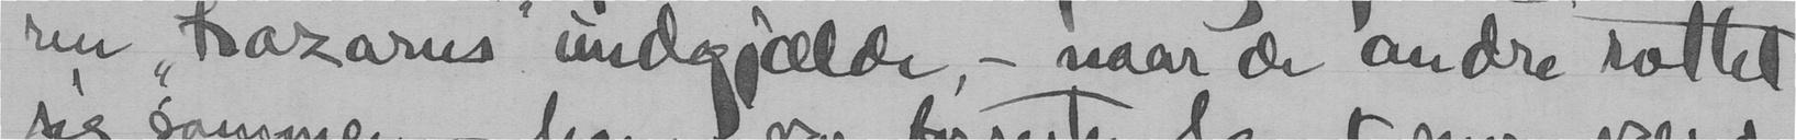ren "Lazarus" undgjælde, - naar de andre rottet
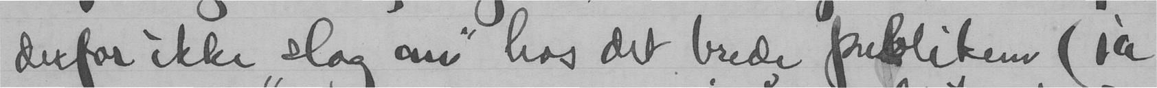derfor ikke "slog av" hos det brede publikum (ja
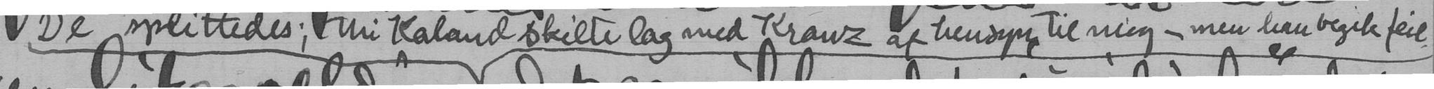De splittedes; thi Kaland skilte lag med Krantz af hensyn til mig - men han begik feil
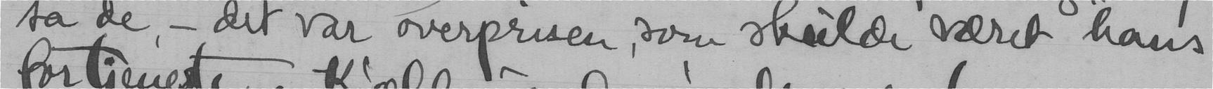sa de, - det var overprisen, som skulde været hans
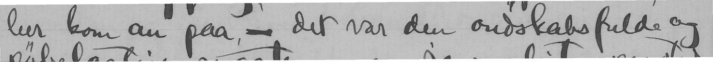her kom an paa, - det var den ondskabsfulde og
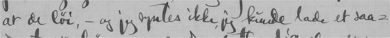at de løi, - og jeg syntes ikke jeg kunde lade et saa-
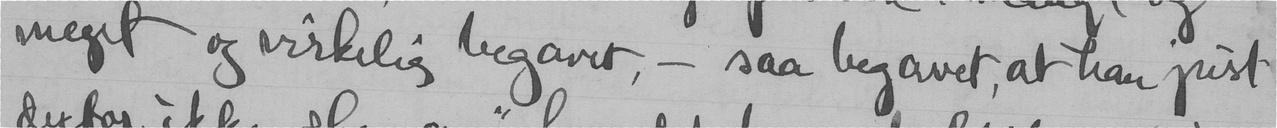meget og virkelig begavet, - saa begavet, at han just
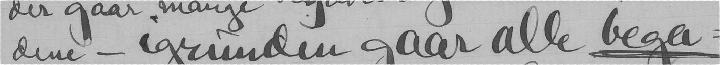dene - igrunden gaar alle bega-
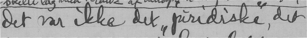det var ikke det "puridiske", det
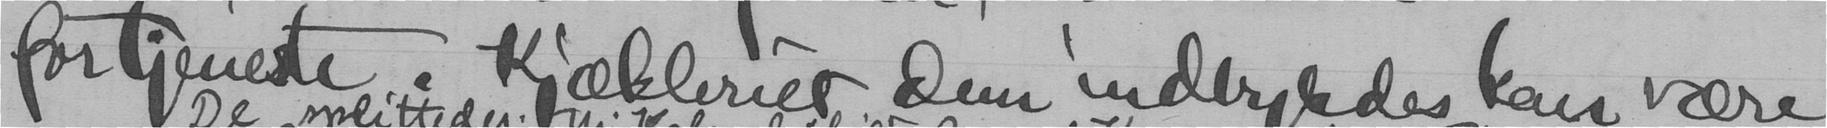fortjeneste. Kjækleriet dem indbyrdes kan være
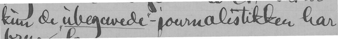kun de "ubegavede" - journalistikken har
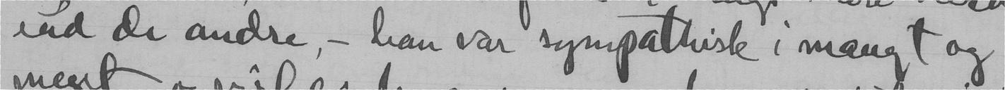end de andre, - han var sympathisk i mangt og
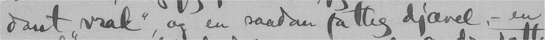dant "vrak", og en saadan fattig djævel, - en
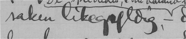saken likegyldig,-
